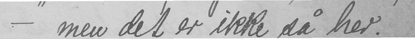- men det er ikke så her.
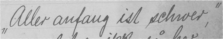"Aller anfang ist schwer,"
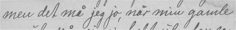men det må jeg jo, når min gamle
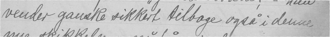vender ganske sikkert tilbage også i denne
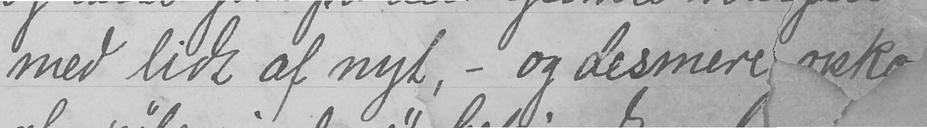med lidt af nyt, - og desmere opkok
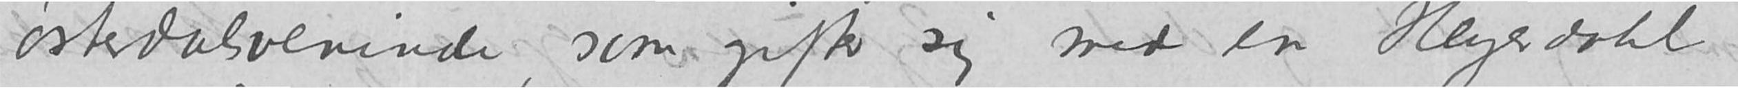østerdalsveninde, som gifter sig med en Heyerdahl
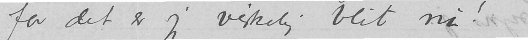for det er jeg virkelig blit nu!
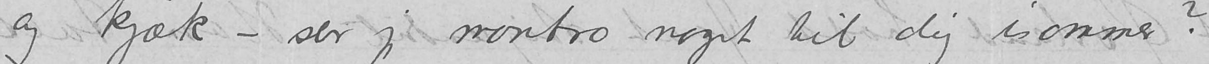og kjæk – ser jeg montro noget til dig isommer?
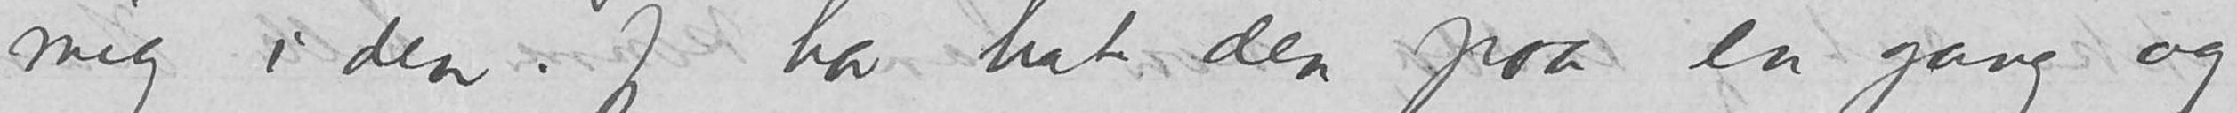mig i den. Jeg har hat den paa en gang og
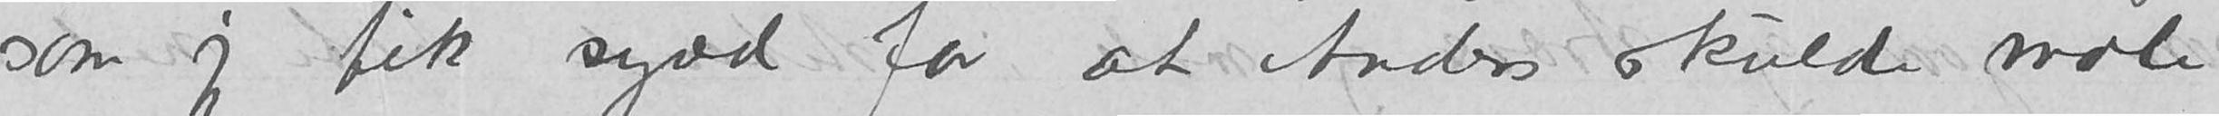som jeg fik sydd for at Anders skulde male
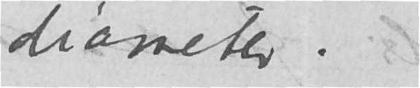diameter. –
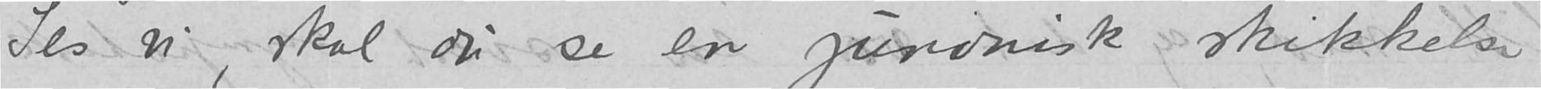Ses vi, skal du se en junonisk skikkelse,
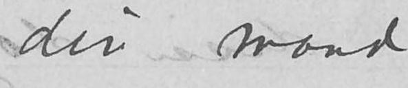mand
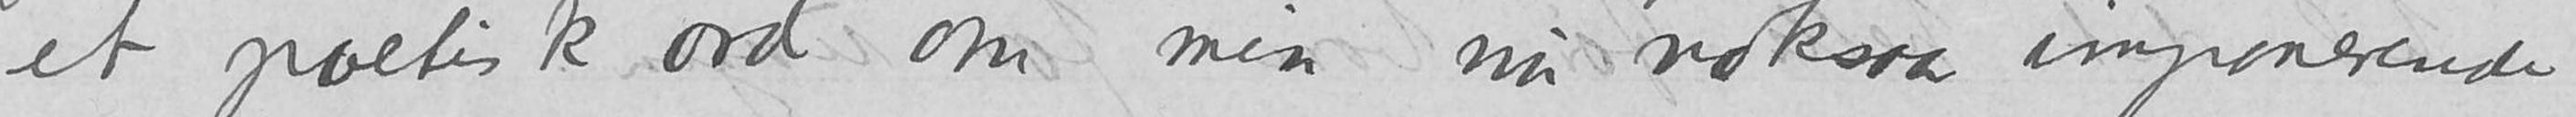et poetisk ord om min nu noksaa imponerende
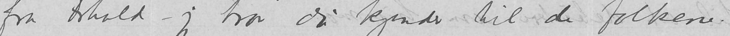fra Frhald ?ald – jeg tror du kjender til de folkene.
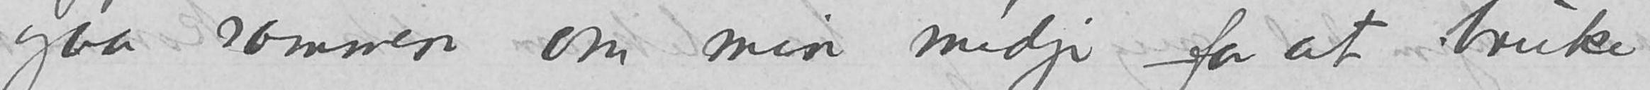gaa sammen om min midje for at bruke
